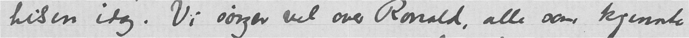hilsen idag. Vi sørger vel over Ronald, alle som kjennte
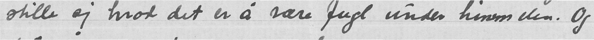stille sig hvad det er å være fugl under himmelen. Og
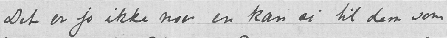Det er jo ikke noe en kan si til dem som
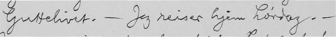Guttelivet. - Jeg reiser hjem Lørdag. -
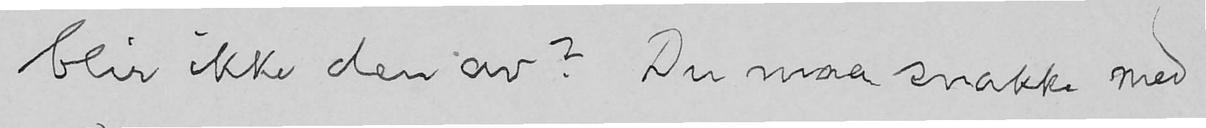blir ikke den av? Du maa snakke med
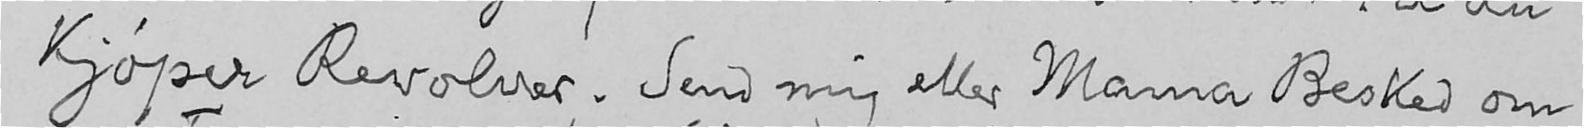kjøper Revolver. Send mig eller Mama Besked om
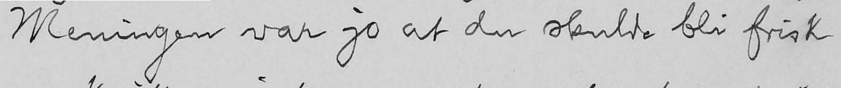Meningen var jo at du skulde bli frisk
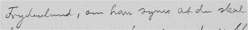Frydenlund, om han synes at du skal
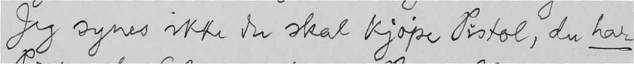Jeg synes ikke du skal kjøpe Pistol, du har
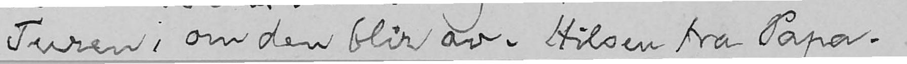Turen; om den blir av. Hilsen fra Papa.
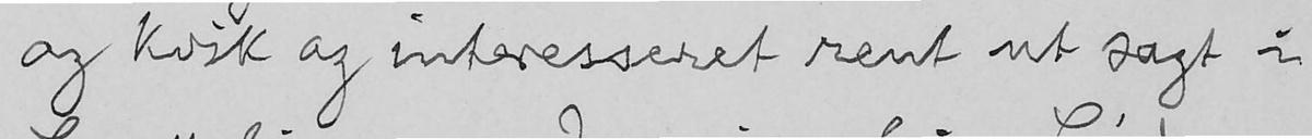og kvik og interesseret rent ut sagt i
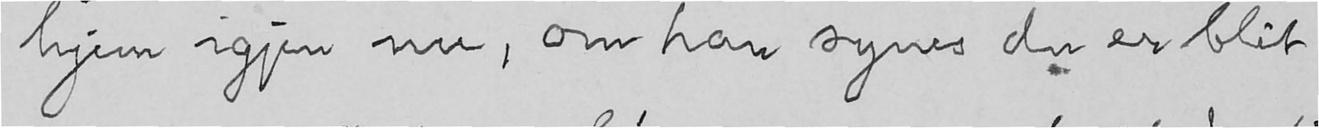hjem igjen nu, om han synes du er blit
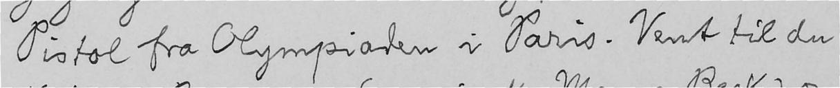Pistol fra Olympiaden i Paris. Vent til du
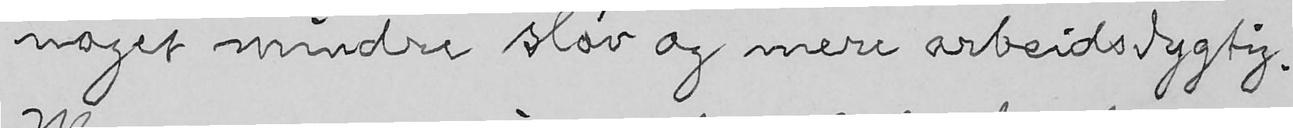noget mindre sløv og mere arbeidsdygtig.
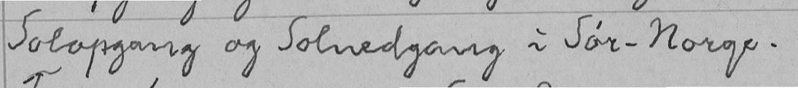Solopgang og Solnedgang i Sør-Norge.
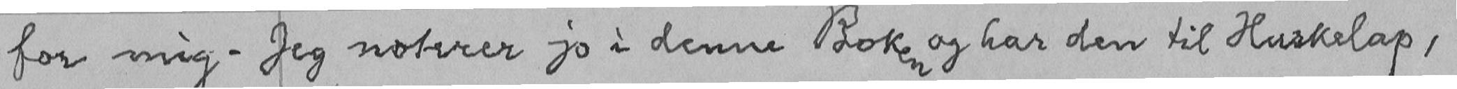for mig. Jeg noterer jo i denne Boken og har den til Huskelap,
